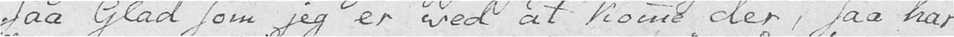saa Glad som jeg er ved at komme der, saa har
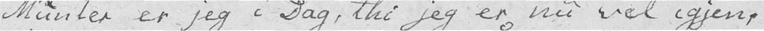Munter er jeg i Dag, thi jeg er nu vel igjen,
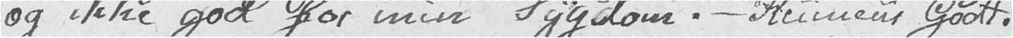og ikke god for min Sygdom. – Humeur Godt.
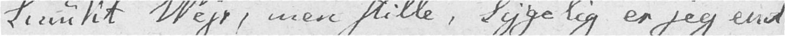Smukt Vejr, men stille, Sygelig er jeg end
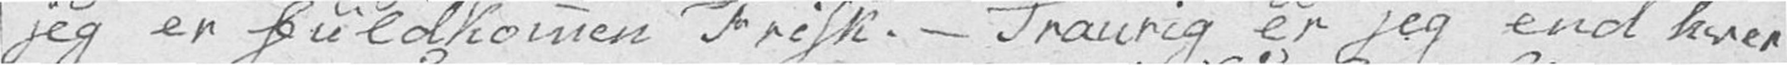jeg er fuldkommen Frisk. – Traurig er jeg end hver
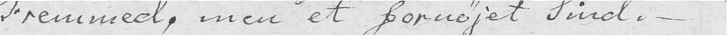Fremmed, men et fornøjet Sind. –
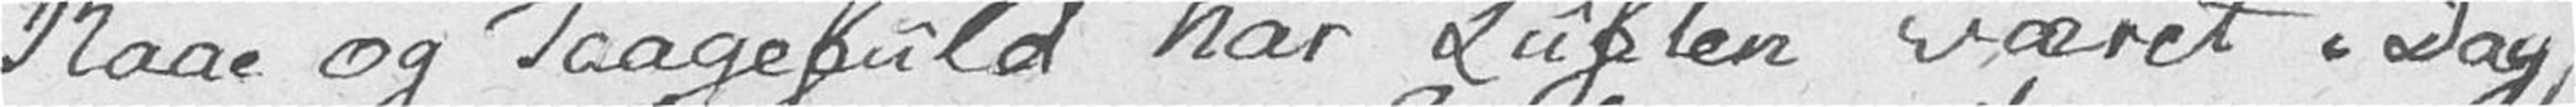Raae og Taagefuld har Luften været i Dag,
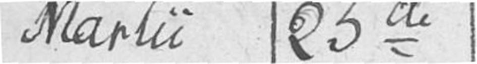Martii 25de
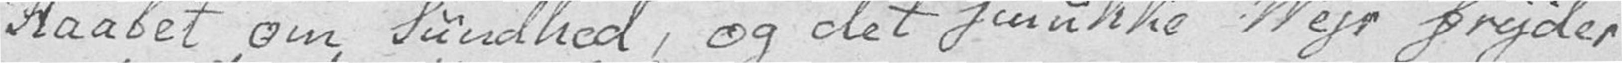Haabet om Sundhed, og det smukke Vejr fryder
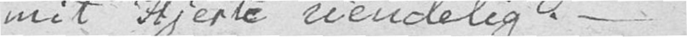mit Hjerte uendelig. –
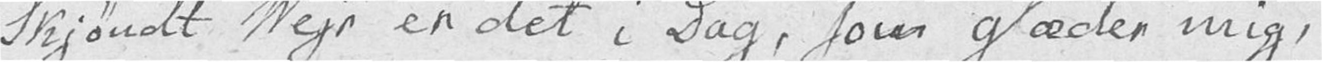Skjøndt Vejr er det i Dag, som glæder mig,
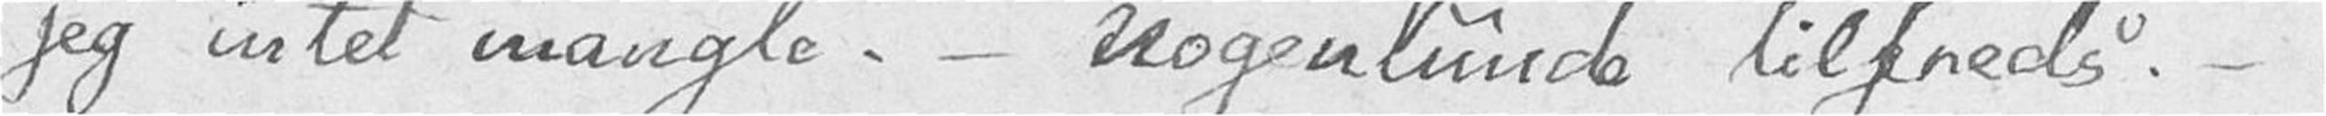jeg intet mangle. – nogenlunde tilfreds. –
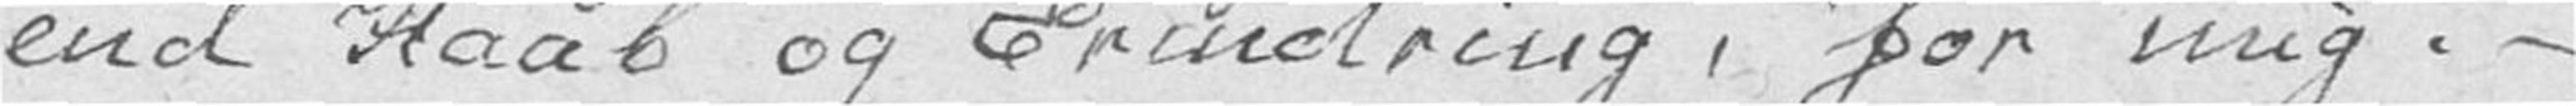end Haab og Erindring, for mig. –
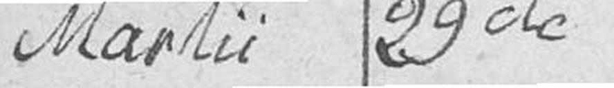Martii 29de
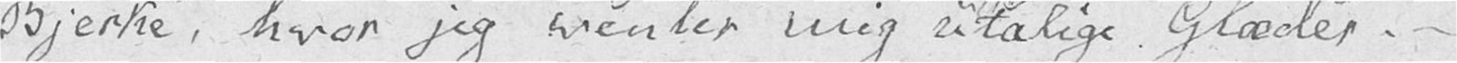Bjerke, hvor jeg venter mig utalige Glæder. –
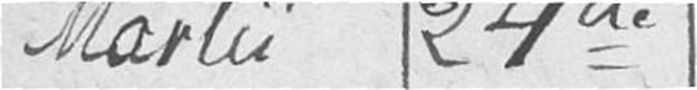Martii 24de
